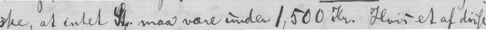ske, at intet Stp. maa være under 1,500 Kr. Hvis et af disse
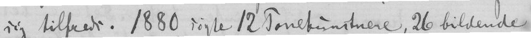sig tilfreds. 1880 søgte 12 Tonekunstnere, 26 bildende
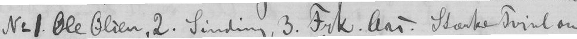No 1 Ole Olsen. 2. Sinding, 3. Frk. Aas. Stærke Tvivl om
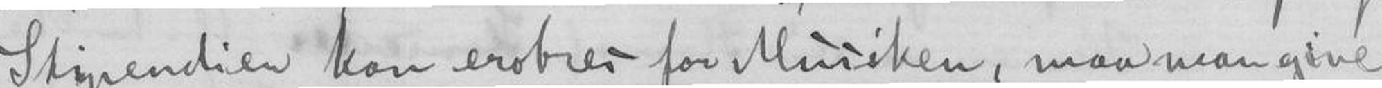Stipendier kan erobres for Musiken, maa man give
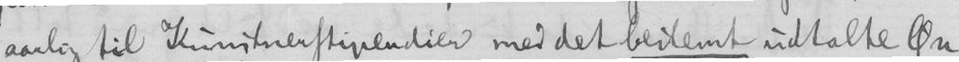aarlig til Kunstnerstipendier med det bestemt udtalte Øn-
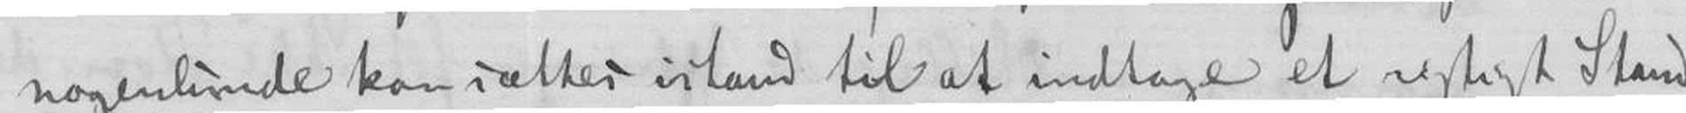nogenlunde kan sættes istand til at indtage et rigtigt Stand-
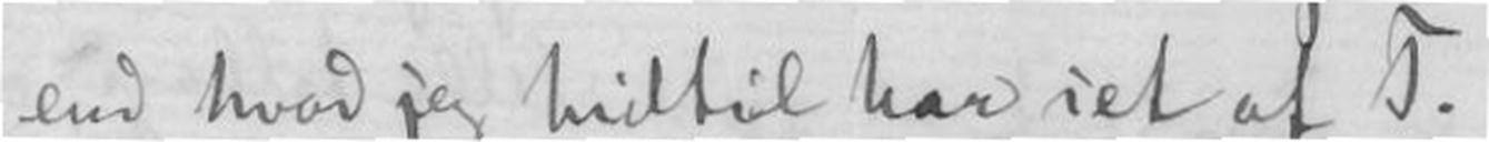end hvad jeg hidtil har set af T.
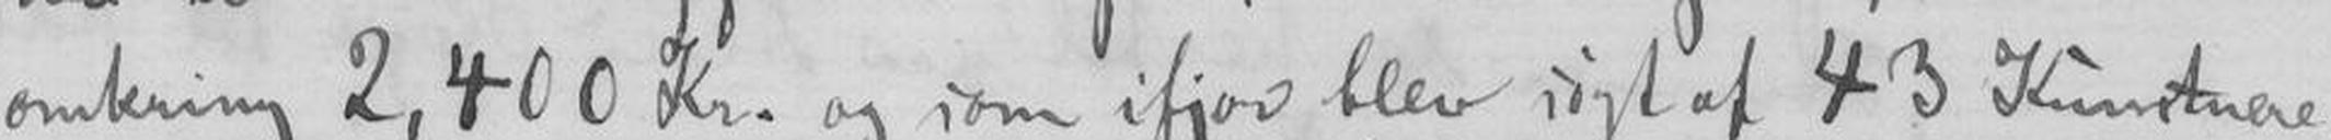omkring 2,400 Kr. og ifjor blev søgt af 43 Kunstnere.
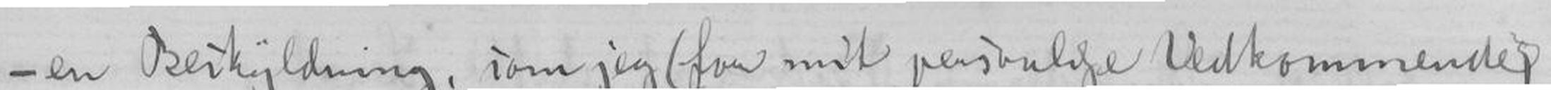- en Beskyldning, som jeg (for mit personlige Vedkommende)
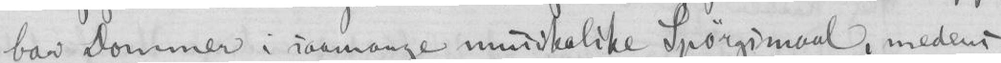bar Dommer i saamange musikalske Spørgsmaal, medens
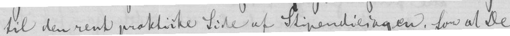til den rent praktiske Side af Stipendiesagen, for at De
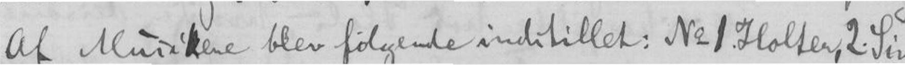Af Musikere blev følgende indstillet: No 1 Holter, 2. Sin-
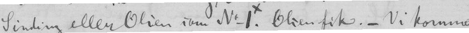Sinding eller Olsen som No 1x. Olsen fik. - Vi kommer
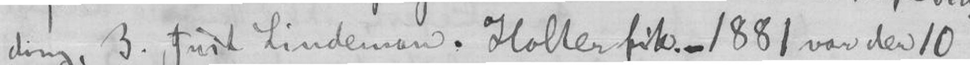ding, 3. Just? Lindeman. Holter fik. - 1881 var der 10
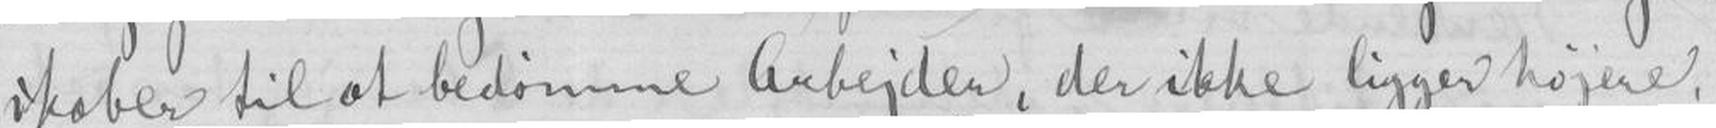skaber til at bedømme Arbejdet, der ikke ligger højere
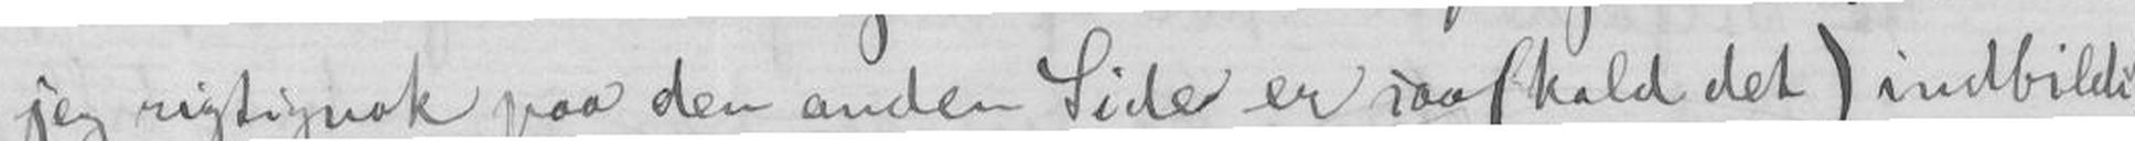jeg rigtignok paa den enden Side er saa (kald det) indbildsk
